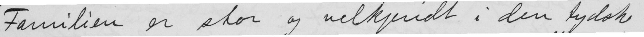Familien er stor og velkjendt i den tydske
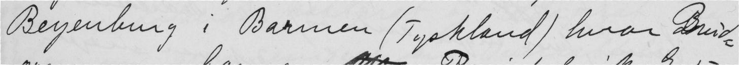Beyenburg i Barmen (Tyskland) hvor Brud-
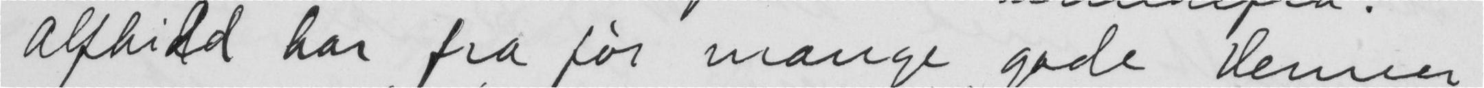Alfhild har fra før mange gode Venner
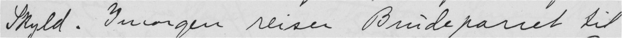Skyld. Imorgen reiser Brudeparret til
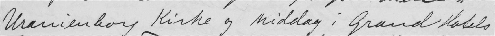Uranienborg Kirke og Middag i Grand Hotels
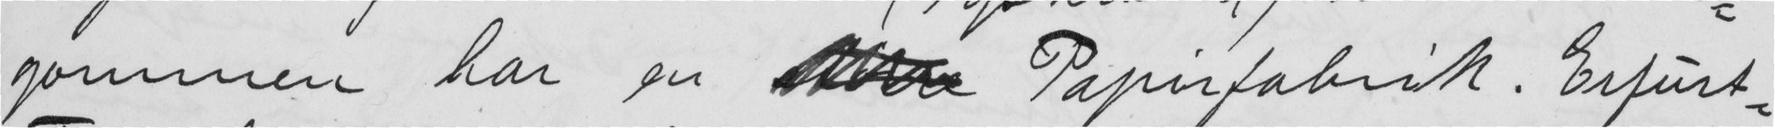gommen har en  Papirfabrik. Erfurt-
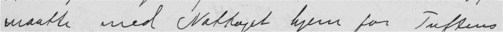maatte med Nattoget hjem for Tuftens
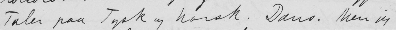Taler paa Tysk og norsk. Dans. Men jeg
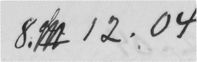8. 12.04
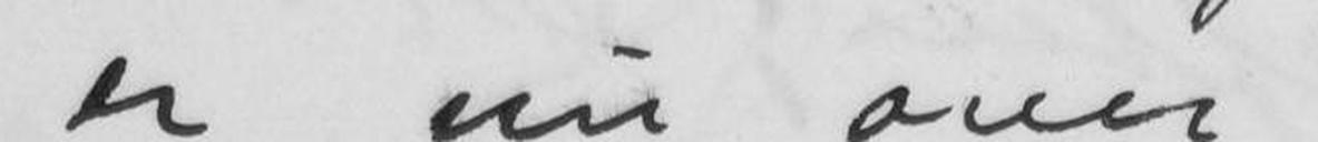er nu over
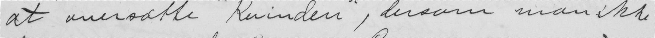at oversætte "Kvinden", dersom man ikke
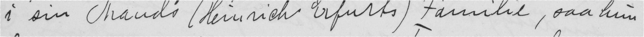i sin Mands (Heinrich Erfurts) Familie, saa hun
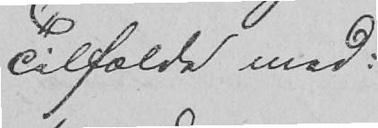Tilfælde med:
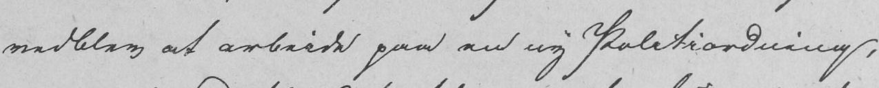vedblev at arbeide paa en ny Politiordning,
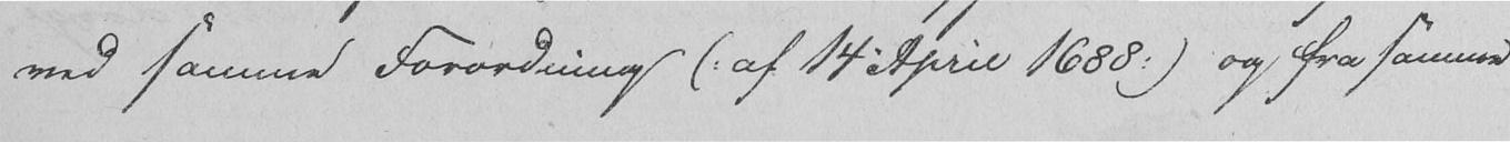ved samme Forordning (: af 14 April 1688 :) og fra samme
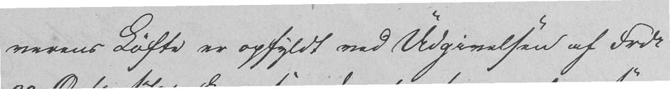verens Løfte er opfyldt ved Udgivelsen af Frdr
